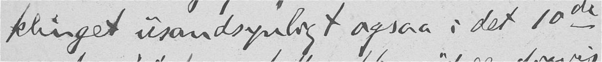klinget usandsynligt ogsaa i det 10de
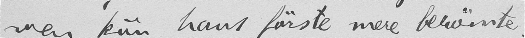men kun hans første mere berømte.
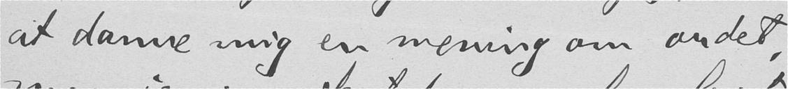at danne mig en mening om ordet,
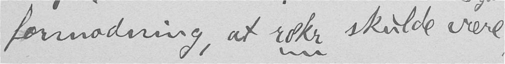formodning, at rækr skulde være
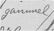gammel
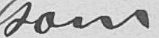som
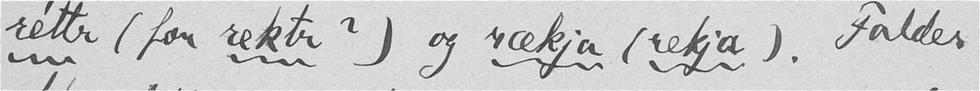réttr (for rektr?) og rækja (rekja). Falder
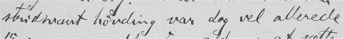stridsvant høvding var dog vel allerede
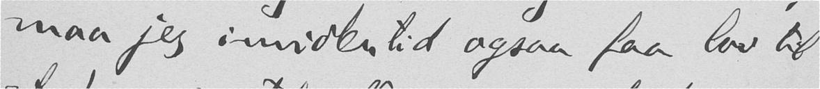maa jeg imidlertid ogsaa faa lov til
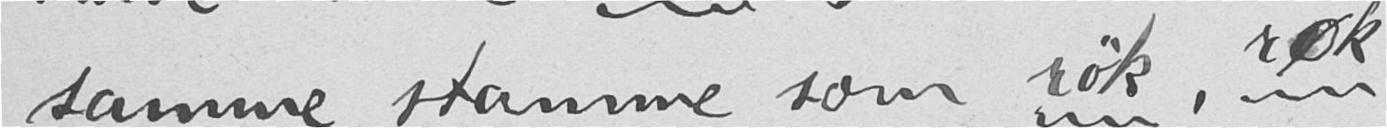samme stamme som rök, røk
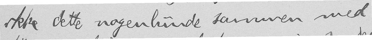ikke dette nogenlunde sammen med
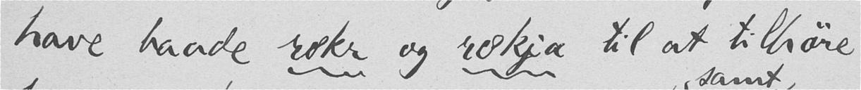have baade rækr og rækja til at tilhøre
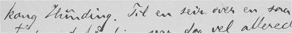kong Hunding. Til en seir over en saa
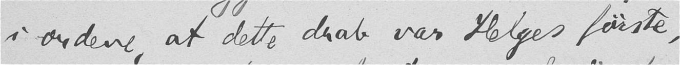i ordene, at dette drab var Helges første,
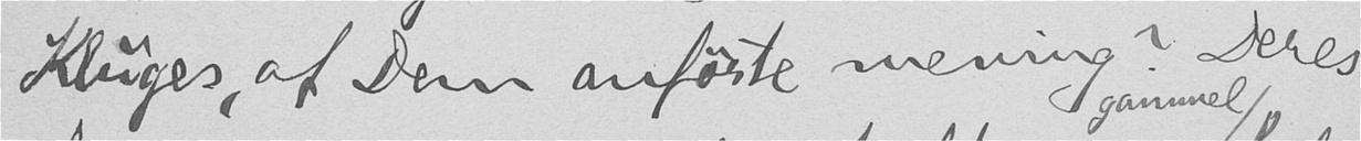Kluges, af Dem anførte mening? Deres
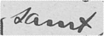samt
